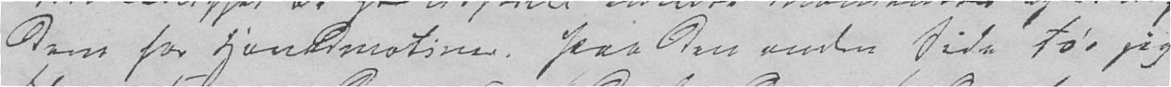dem for Hovedmotiver. Paa den anden Side tør jeg
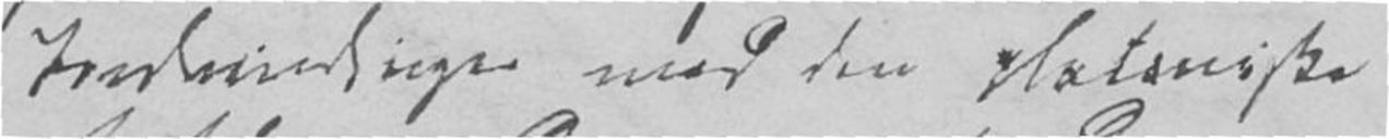Indvendinger mod den platoniske
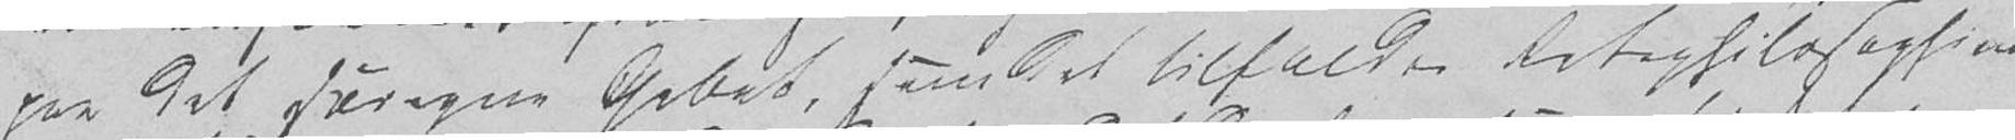paa det særegne Gebet, som det tilfalder Retsphilosophien
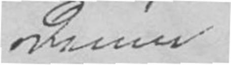Denne
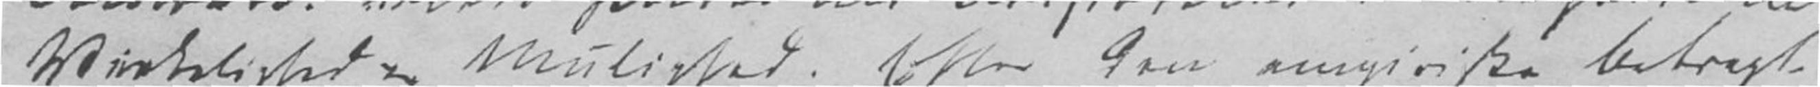Virkelighed og Mulighed. Efter den empiriske Betragt-
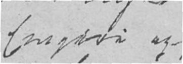Empiri og
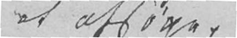at afsøge,
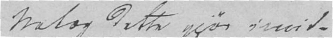Netop dette gjør imid-
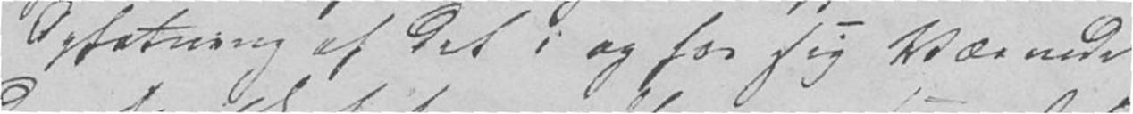Opfatning af det i og for sig Værende
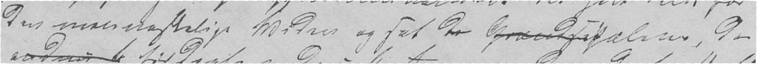den menneskelige Viden og sat de GrundPælene, der
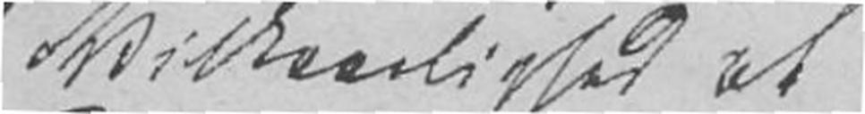Vilkaarlighed at
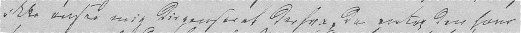ikke ansee mig dispenseret derfra, da netop den hans
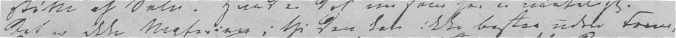Det er ikke Materien; thi den kan ikke bestaa uden Form,
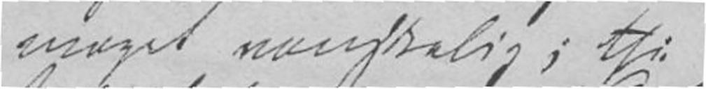meget vanskelig; thi
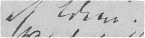af Ideen.
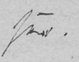ser.
